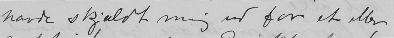havde skjældt mig ud for et eller
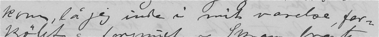kom, lå jeg inde i mit værelse, for-
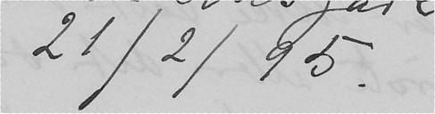21/2/95.
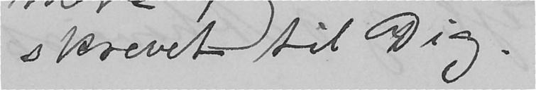skrevet til Dig.
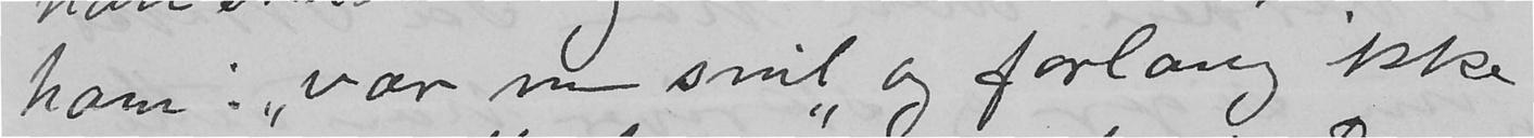ham: "vær nu snil og forlang ikke
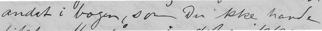andet i bogen, som Du ikke havde
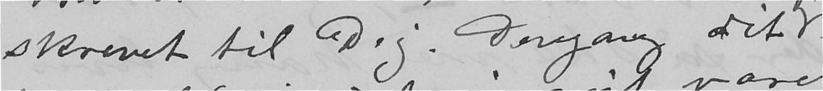skrevet til Dig. Dengang dit
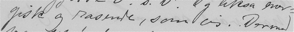gisk og rasende, som jeg. Dermed
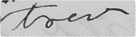brev
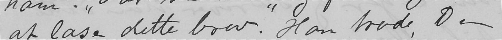at læse dette brev." Han trode, Du
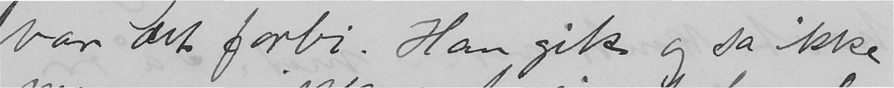var det forbi. Han gik og sa ikke
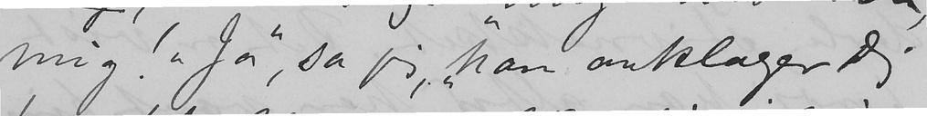mig!" "Ja", sa jeg, kan anklager dig
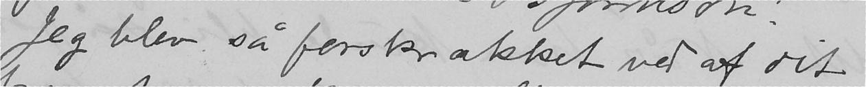Jeg blev så forskrækket ved af dit
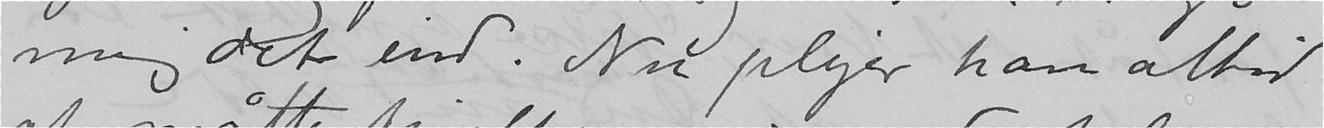mig det ind. Nu plejer han altid
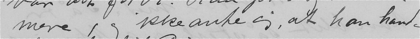mere, og ikke ante jeg, at han havde
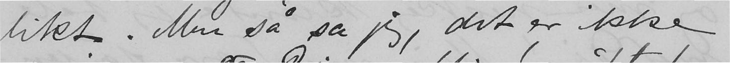likt. Men så sa jeg, det er ikke
